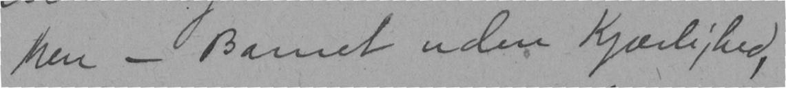Men - Barnet uden Kjærlighed,
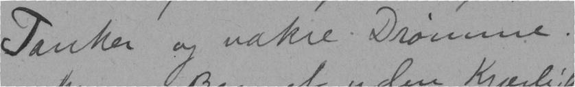Tanker og vakre Drømme.
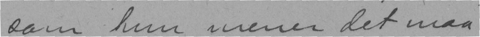som hun mener det maa
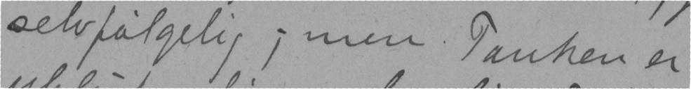selvfølgelig; men. Tanken er
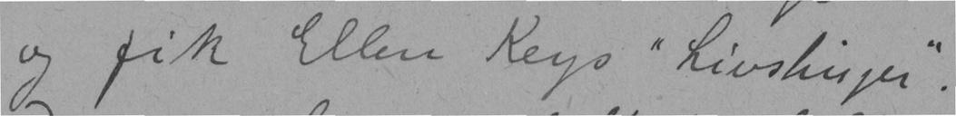og fik Ellen Keys "Livslinjer".
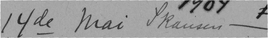14de mai Skansen -
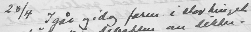28/4 Igår og i dag form. i stortinget
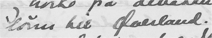lønn til Øverland.
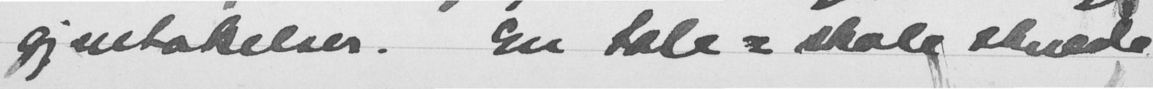gjentakelser. En tale-skole skulde
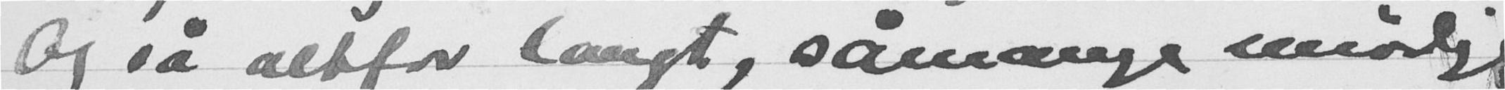Og så altfor langt, såmange unødige
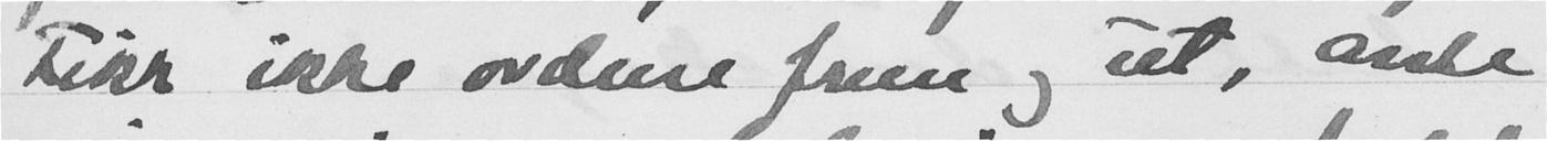Fikk ikke ordene frem og ut, ante
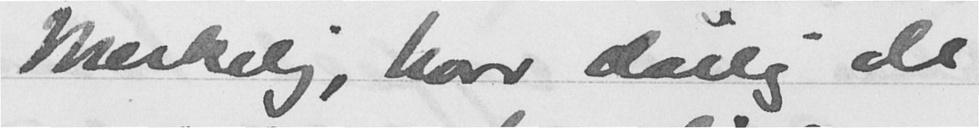Merkelig, hvor dårlig de
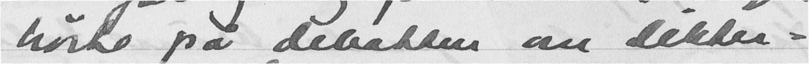og hørte på debatten om dikter-
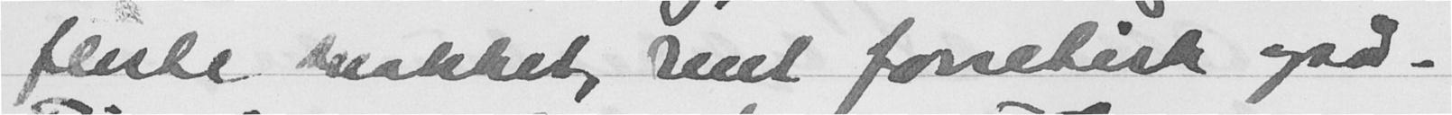fleste snakket rent fonetisk også.
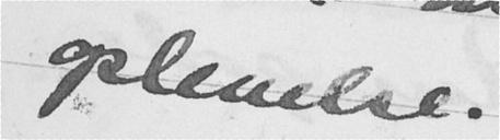oplevelse.
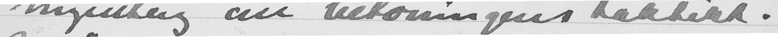ingenting om betoningens taktikk.
